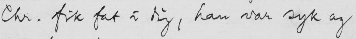Chr. fik fat i dig, han var syk og
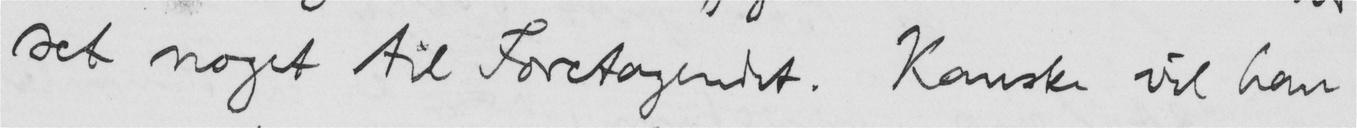set noget til Foretagendet. Kanske vil han
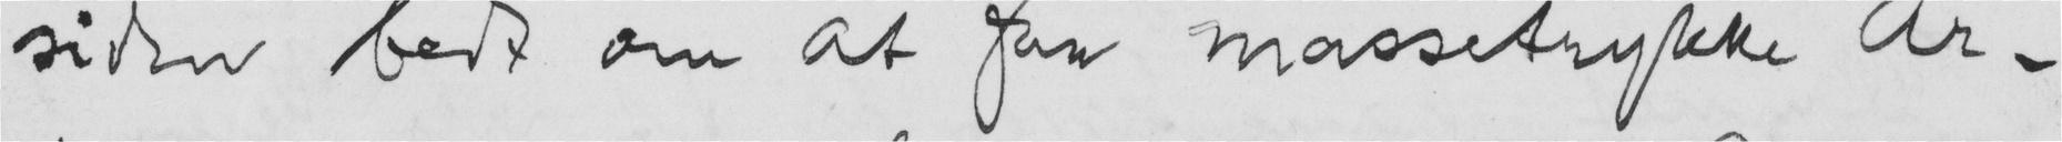siden bedt om at faa massetrykke Ar-
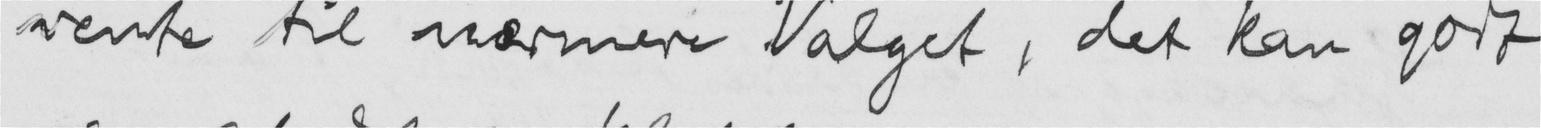vente til nærmere Valget, det kan godt
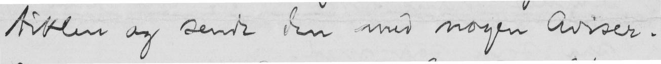tiklen og sende den med nogen Aviser.
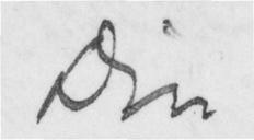Din
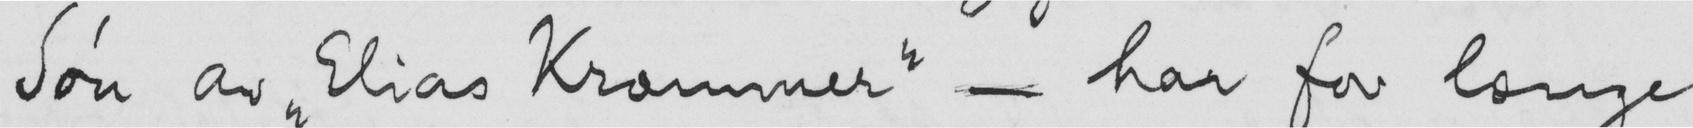Søn av "Elias Kræmmer" - har for længe
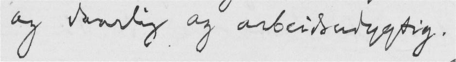og daarlig og arbeidsudygtig.
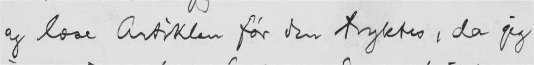og læse Artiklen før den tryktes, da jeg
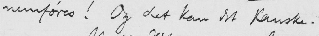nemføres! Og det kan det kanske.
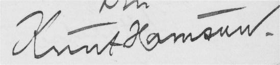Knut Hamsun.
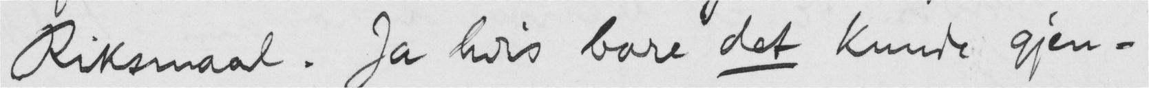Riksmaal. Ja hvis bare det kunde gjen-
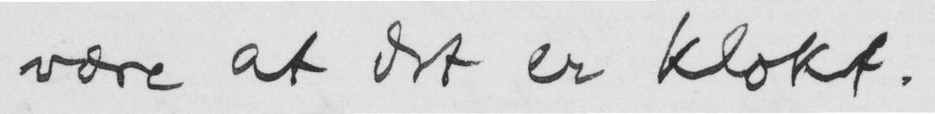være at det er klokt.
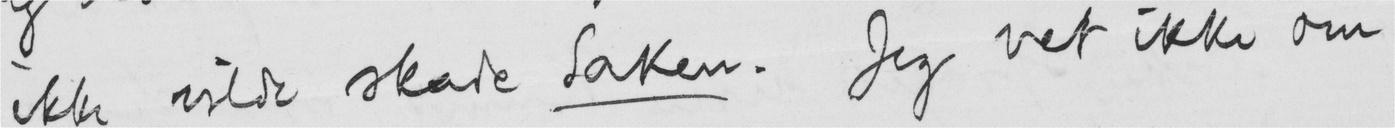ikke vilde skade Saken. Jeg vet ikke om
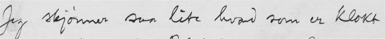Jeg skjønner saa lite hvad som er klokt
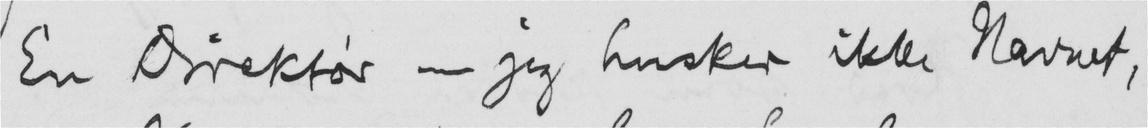En Direktør - jeg husker ikke Navnet,
